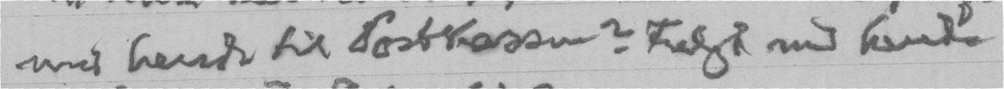med hende til Postkassen? Fulgt med hende
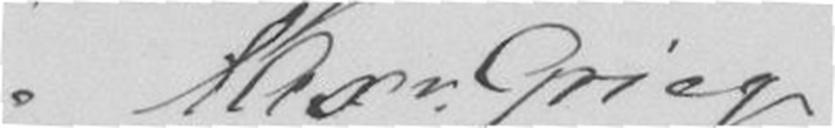Alexr. Grieg
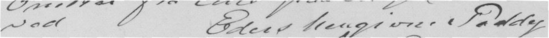ved Eders hengivne Paddy
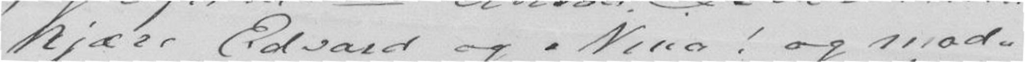kjære Edvard og Nina! og mod-
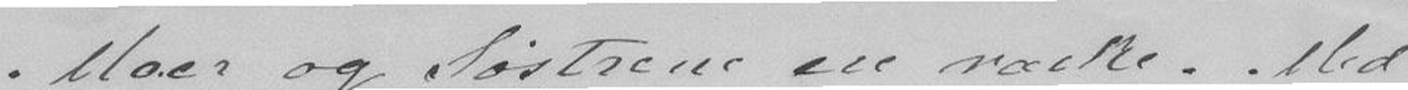Moer og Søstrene ere raske. Med
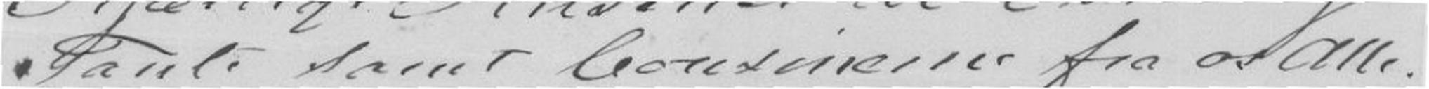Tante samt Cousinerne fra os Alle.
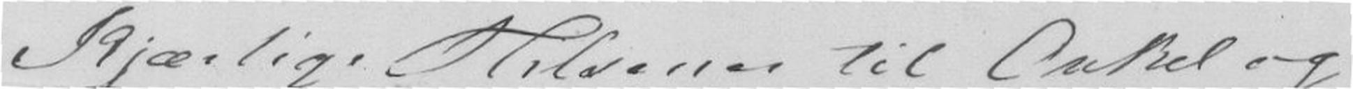Kjærlige Hilsener til Onkel og
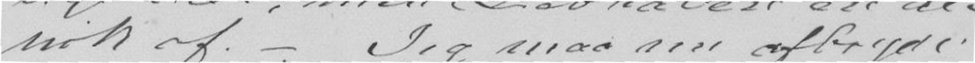nok af. - Jeg maa nu afbryde
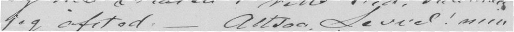jeg afsted - Altsaa. Level! min
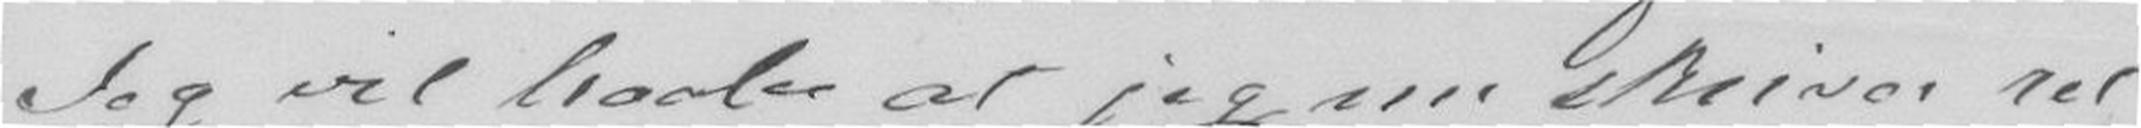Jeg vil haabe at jeg nu skriver ret
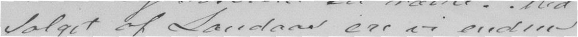Salget af Landaas ere vi endnu
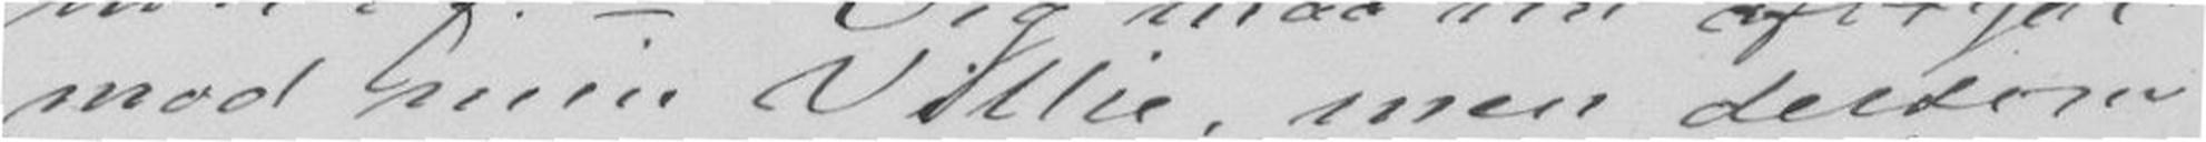mod min Villie, men dersom
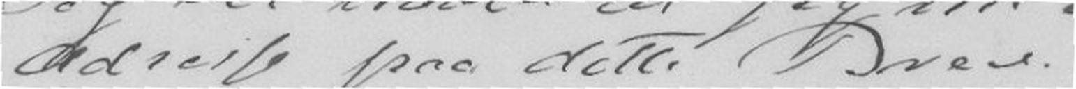Adresse paa dette Brev.
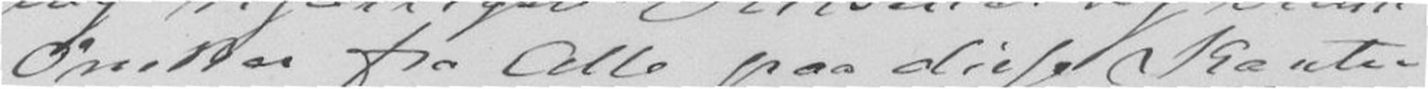Ønsker fra Alle paa disse Kanter
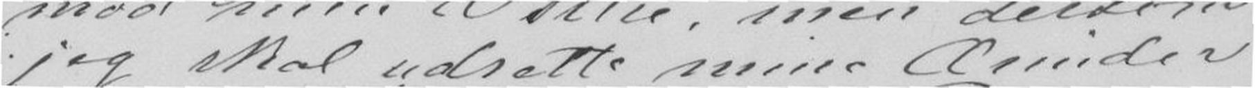jeg skal udsette mine Ærinder
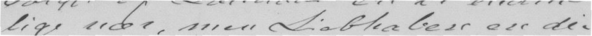lige nær, men Liebhabere ere der
